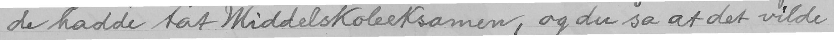de hadde tat Middelskoleeksamen, og du sa at det vilde
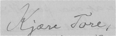Kjære Tore,
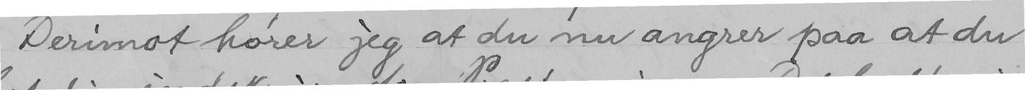Derimot hører jeg at du nu angrer paa at du
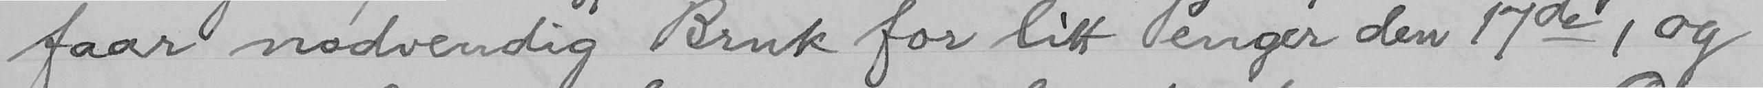faar nødvendig Bruk for litt Penger den 17de, og
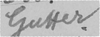Gutter
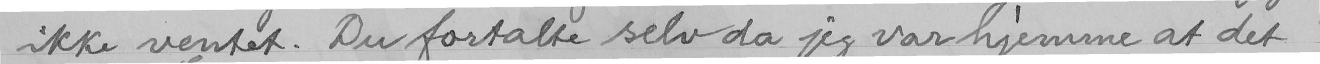ikke ventet. Du fortalte selv da jeg var hjemme at det
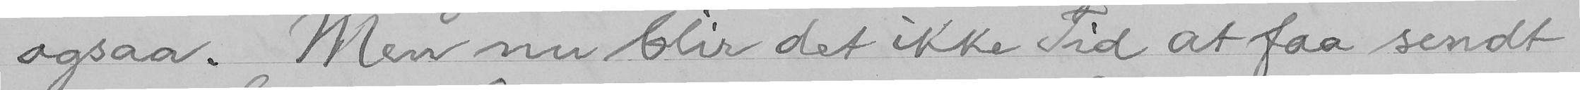ogsaa. Men nu blir det ikke Tid at faa sendt
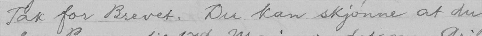Tak for Brevet. Du kan skjønne at du
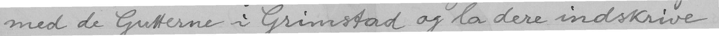med de Gutterne i Grimstad og la dere indskrive
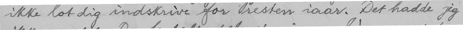ikke lot dig indskrive for Presten iaar. Det hadde jeg
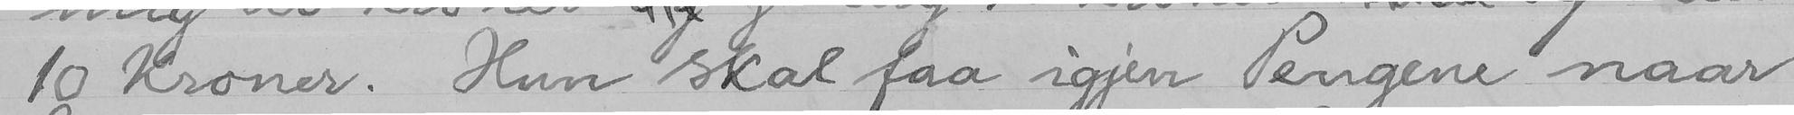10 Kroner. Hun skal faa igjen Pengene naar
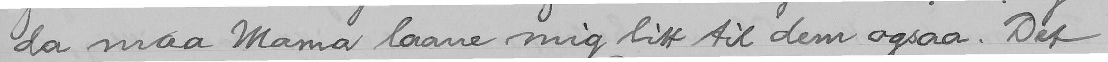da maa Mama laane mig litt til dem ogsaa. Det
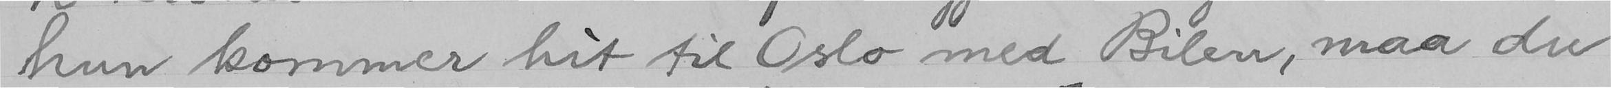hun kommer hit til Oslo med Bilen, maa du
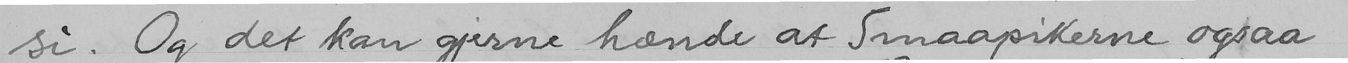si. Og det kan gjerne hænde at Smaapikerne ogsaa
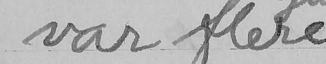var flere
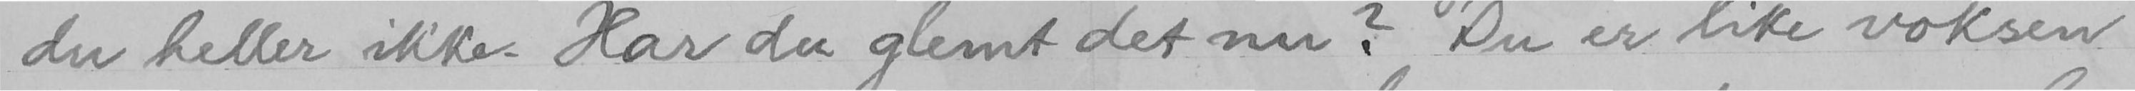du heller ikke. Har du glemt det nu? Du er like voksen
